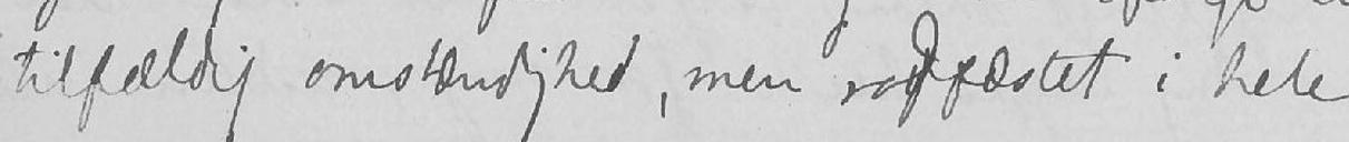tilfældig omstendighed, men rodfæstet i hele
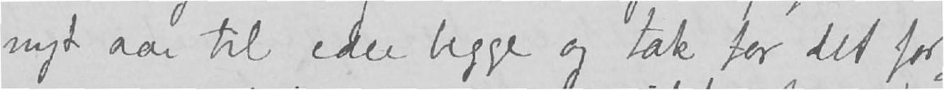nyt aar til eder begge og tak for det for-
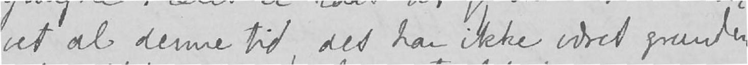vet al denne tid, det har ikke været grunden
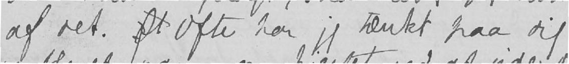af det. Øt Ofte har jeg tænkt paa dig
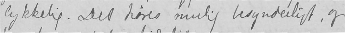lykkelig. Det høres mulig besynderligt, og
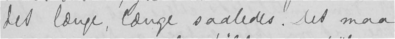det længe, længe saaledes. Det maa
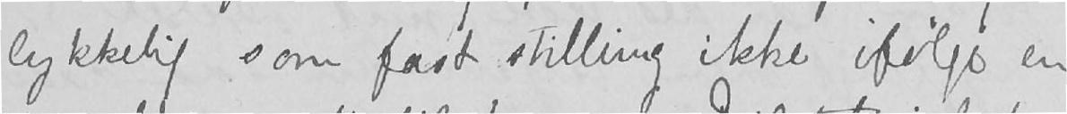lykkelig som fast stilling ikke ifølge en
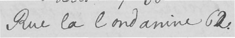Rue la Condanine 62.
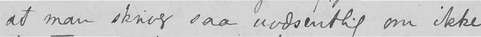at man skriver saa uvæsentlig om ikke
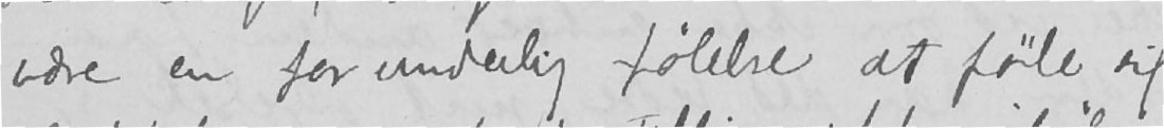være en forunderlig følelse at føle sig
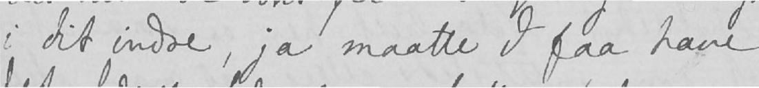i dit indre, ja maatte I faa have
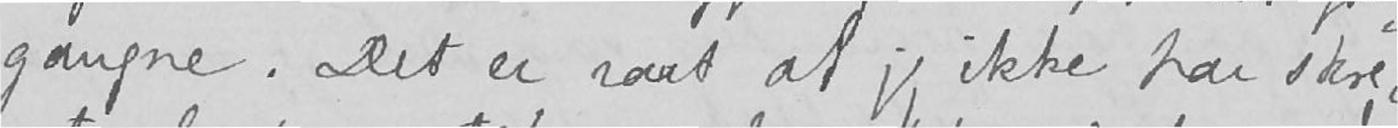gangne. Det er rart at jeg ikke har skre-
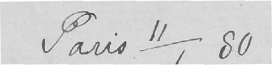Paris 11/1 80
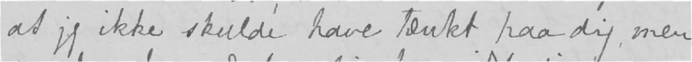at jeg ikke skulde have tænkt paa dig, men
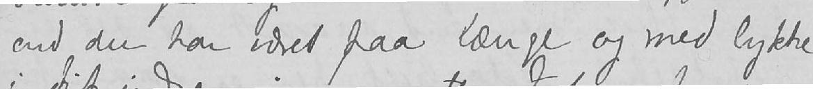end du har været paa længe og med lykke
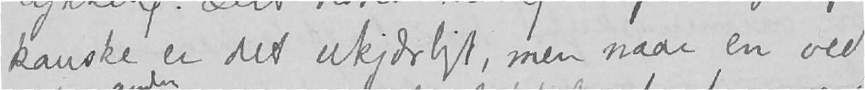kanske er det ukjærligt, men naar en ved,
